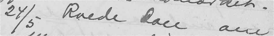24/5 Roede Don om
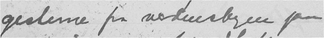gesterne fra verdensbyen paa
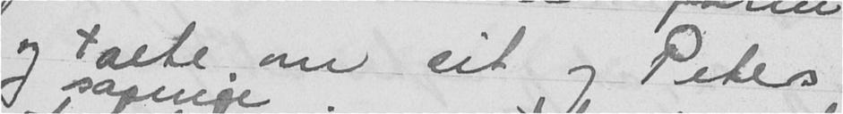og talte om sit og Peters
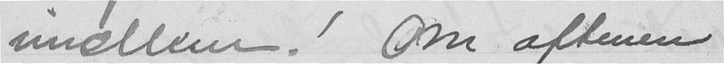imellem! Om aftenen
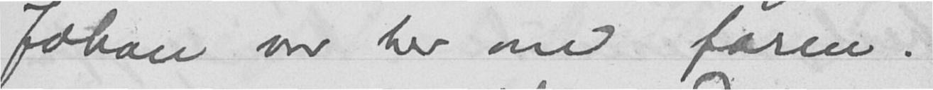Johan var her om form.
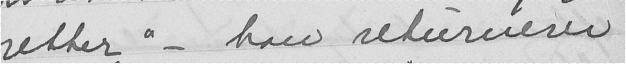retter" - han returnerer
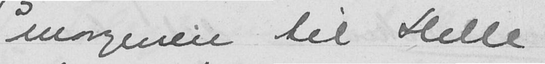morgenen til Helle
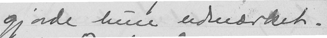gjorde hun udmærket.
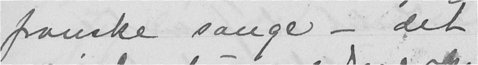franske sange - det
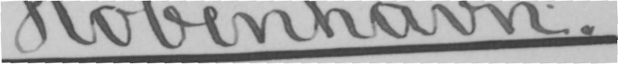København.
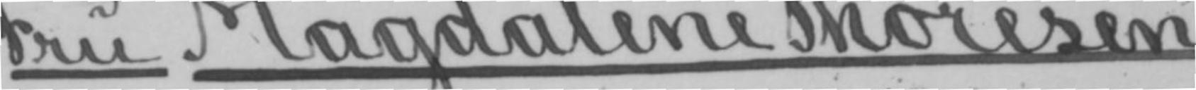Fru Magdalene Thoresen,
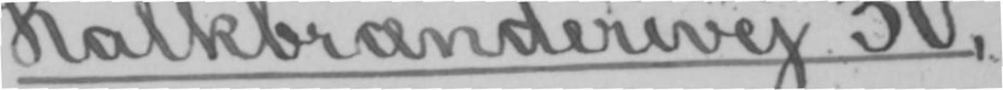Kalkbrænderivej 30,
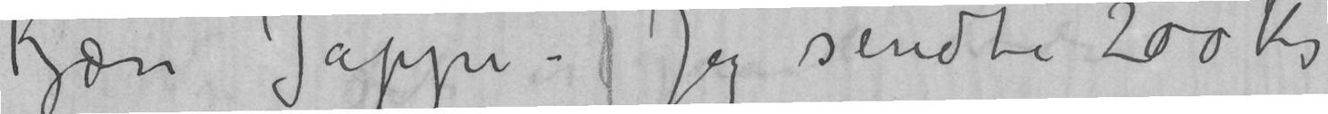Kjære Jappe – Jeg sendte 200 Kr
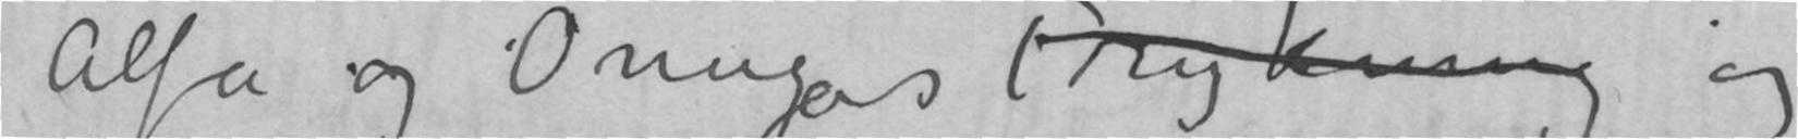Alfa og Omegas Trykning og
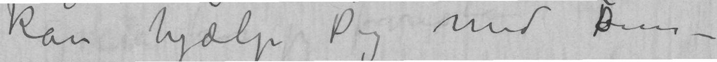kan hjælpe Dig med Dem –
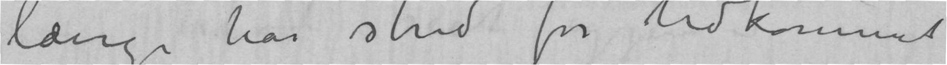længe har Strid for Udkommet
